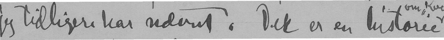jeg tidligere har nævnt. Det er en historie
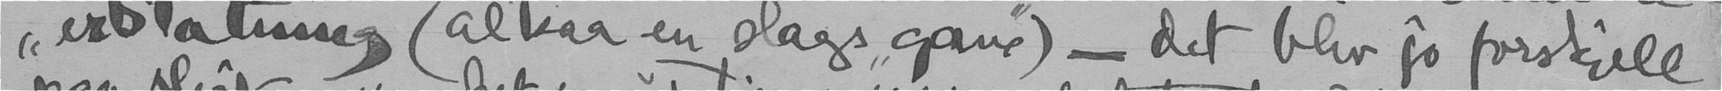"erstatning (altsaa en slags "gave") - det blev jo forskjell
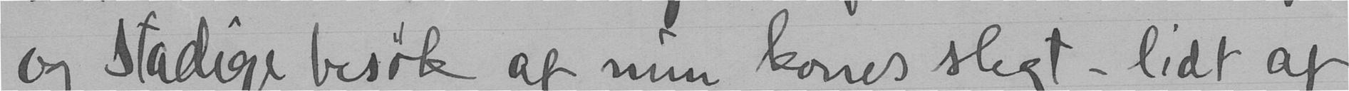og stadige besøk af min kones slegt - lidt af
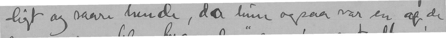ligt og saare hende, da hun ogsaa var en af de
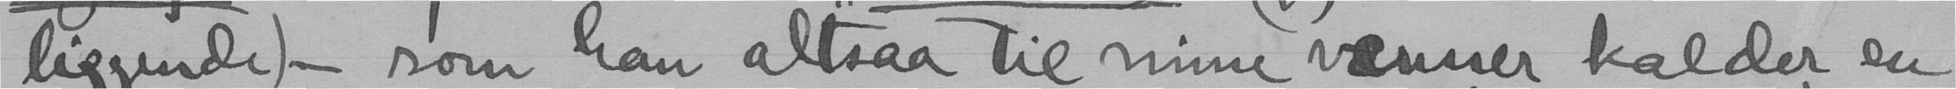liggende) - som han altsaa til mine venner kalder en
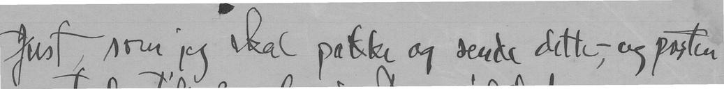Just, som jeg skal pakke og sende dette, - og posten
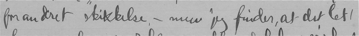forandret skikkelse, - men jeg finder, at det lett
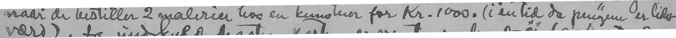naar de bestiller 2 malerier hos en kunstner for kr. 1000. (i en tid da pengene er lidet
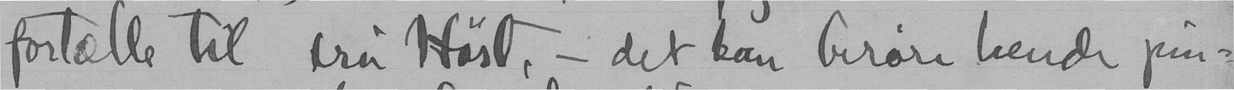fortælle til fru Høst, - det kan berøre hende pin-
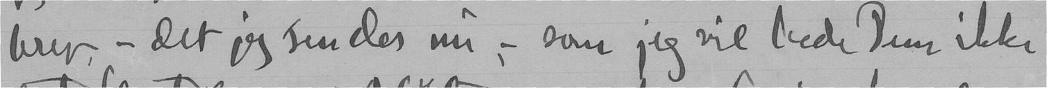brev, - det jeg sender nu, - som jeg vil bede Den ikke
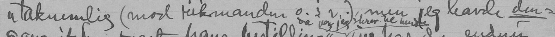utaknemlig (mod rikmanden o.s v.), men jeg havde den-
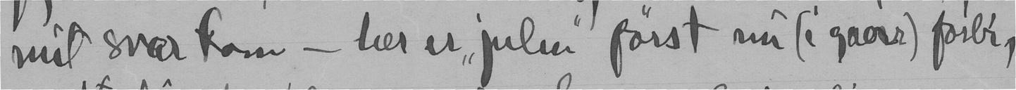mit svar kom - her er "julen" først nu (i gaarr) forbi,
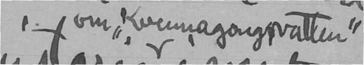om "Kvennagongsvatten"
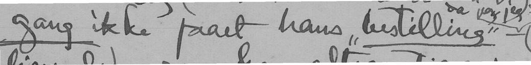gang ikke faaet hans "bestilling". -
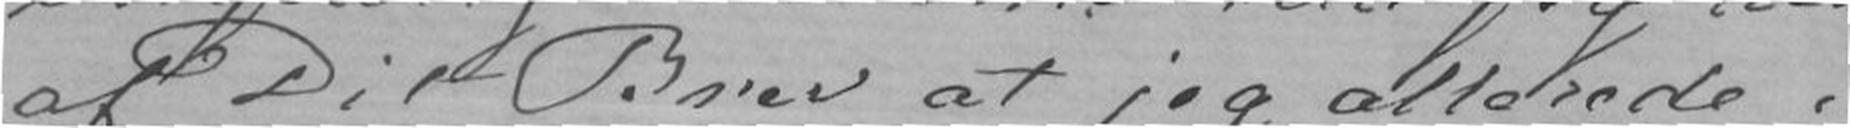af Dit Brev at jeg allerede i
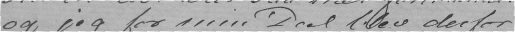og jeg for min Deel blev derfor
